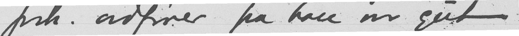forh. ordfører fra bare en gut.
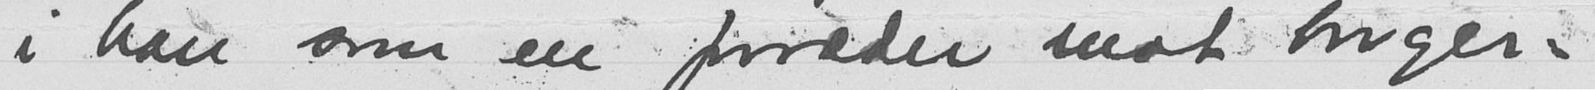i ban som en forræder mot
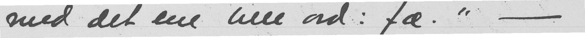med det ene lille ord: fæ.” -
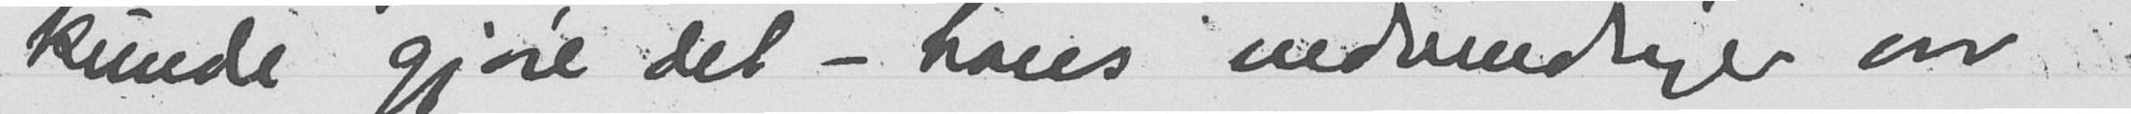kunde gjøre det - hans indvendinger var
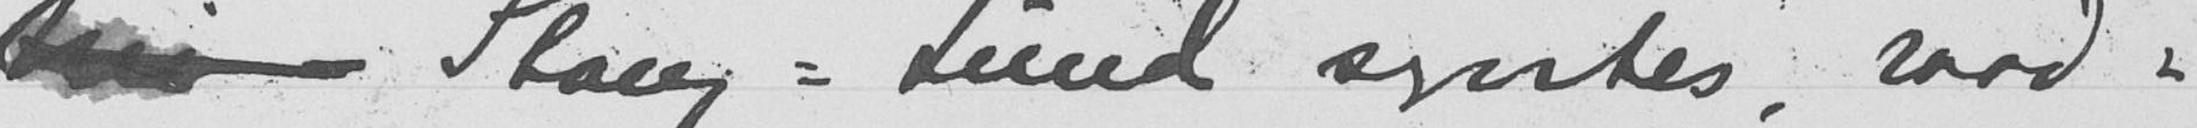Stang-Lund syntes, raad-
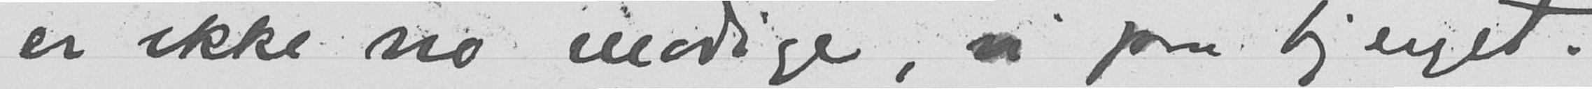er ikke no modige, vi paa bjerget.
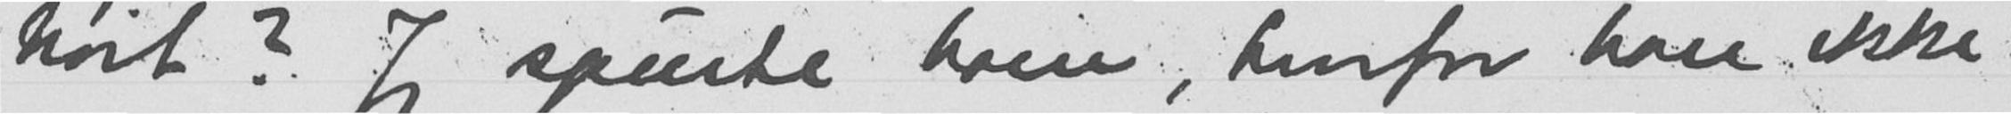høit? Jeg spurte ham, hvorfor han ikke
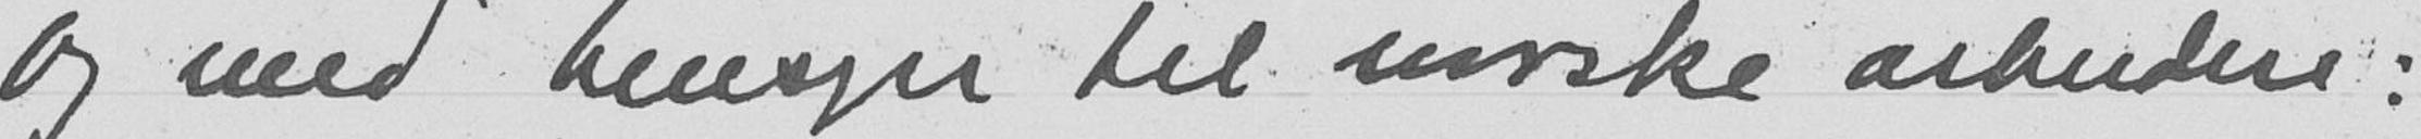Og med hensyn til norske arbeidere:
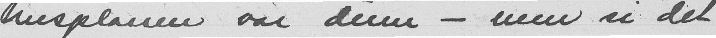husplanen var dum — men si det
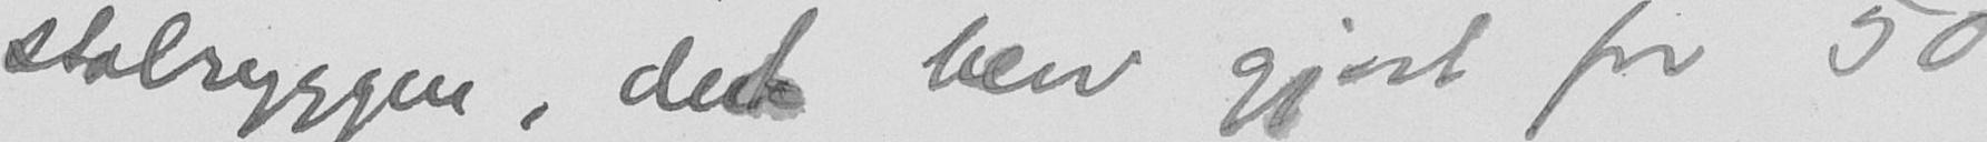stolryggen, det blev gjort for 50
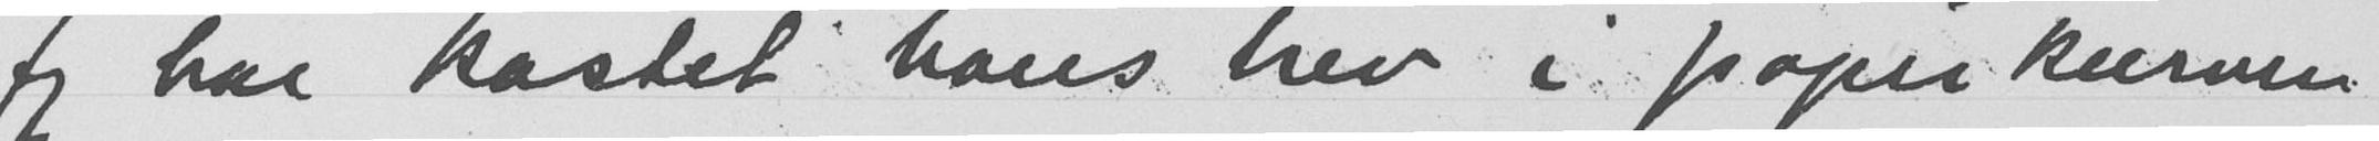Jeg har kastet hans brev i papirkurven
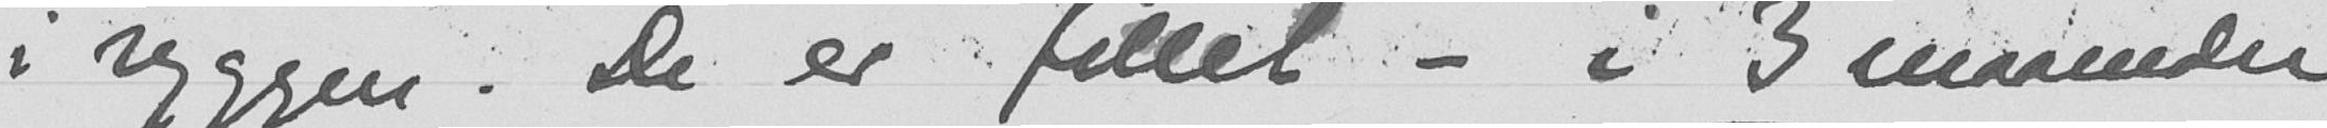i ryggen. De er fillet - i 3 maaneder
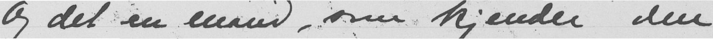Og det en mand, som kjender den
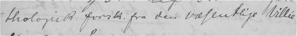thologisk forsk. fra den væsentlige Villie
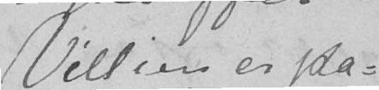Villien er pa-
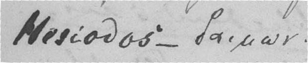Hesiodos - Januar.
